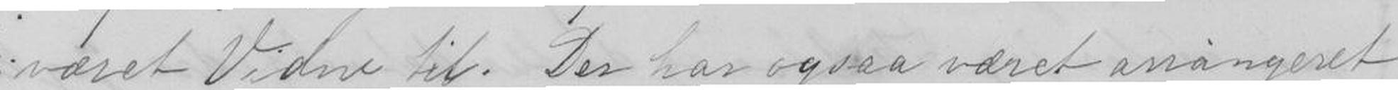været Vidne til. Der har ogsaa været arrangeret
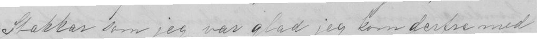Stakkar som jeg var glad jeg kom derfra med
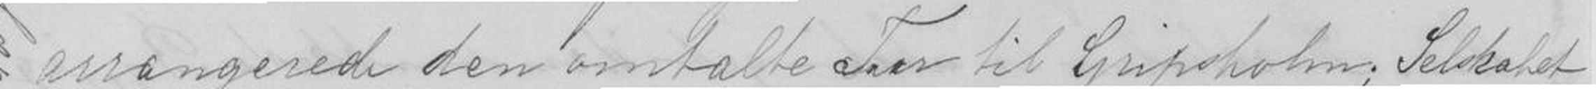arrangerede den omtalte Tur til Gripholm; Selskabet;
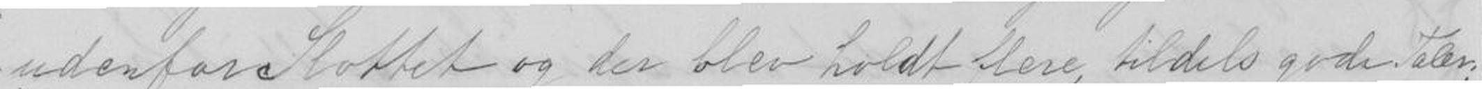udenfor Slottet og der blev holdt flere, tildels gode Taler;
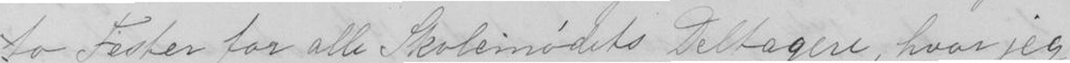to fester for alle Skolemødets Deltagere, hvor jeg
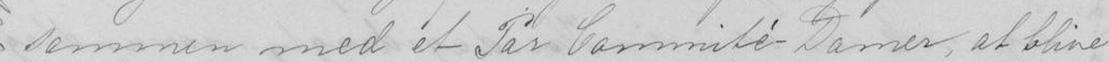sammen med et Par Commite'- Damer, at blive
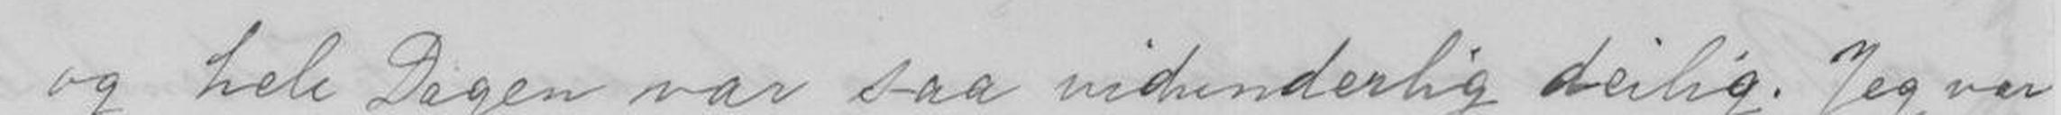og hele dagen var saa vidunderlig deilig. jeg var
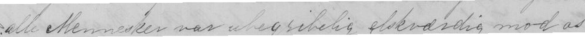alle Mennesker var Ubegribelig elskværdig mot os
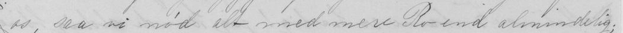os, saa vi nød alt med mere Ro end almindelig;
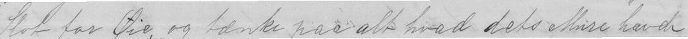Slot for Øie, og tænke paa alt hvad dets Mine havde
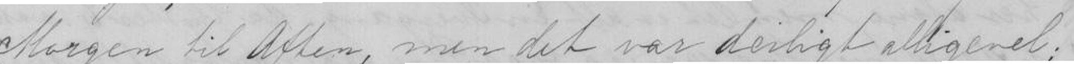Morgen til Aftens, men det var deiligt alligervel;
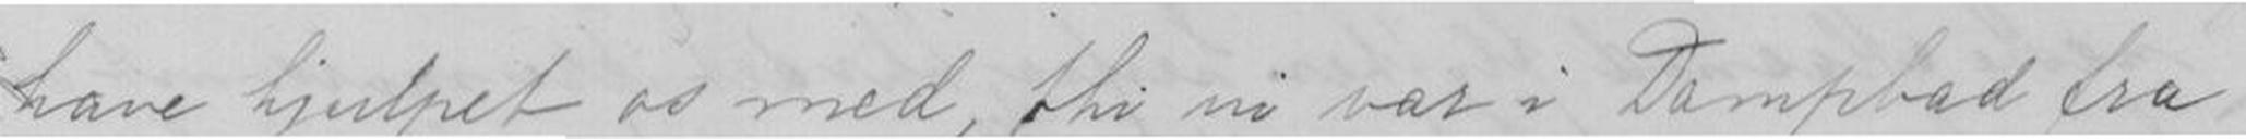have hjulpet os med, thi vi var i Dampbad fra
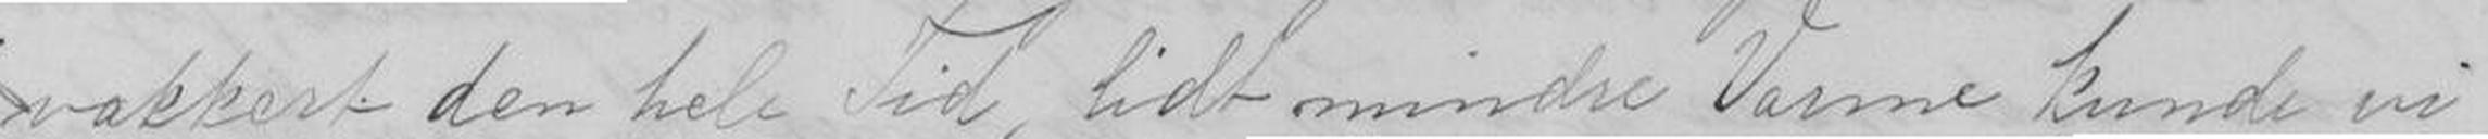vakkert den heletid, lidt mindre Varme kunde vi
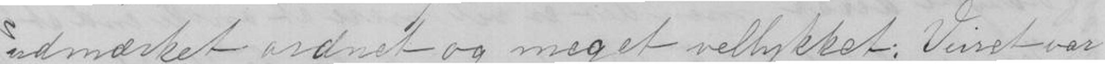udmærket ordnet og meget vellykket. Visst var
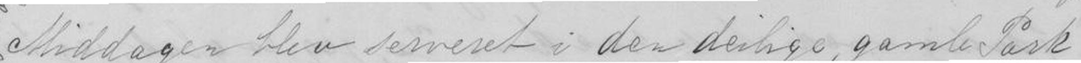Middagen blev servert i den dilige, gamle Park
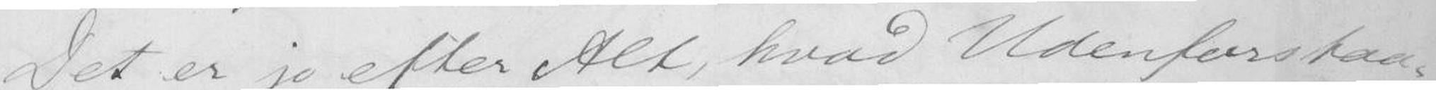Det er jo efter Alt, hvad Udenforstaa-
